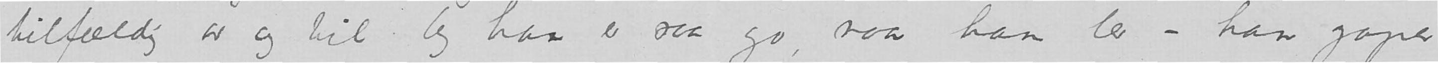tilfældig av og til. Oog han er saa go, naar han ler – han gaper
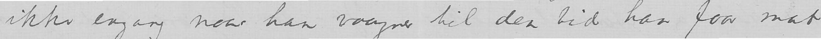ikke engang naar han vaagner til den tid han faar mat
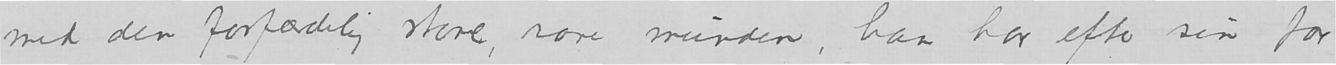med den forfærdelig store, rare munden, han har efter sin far
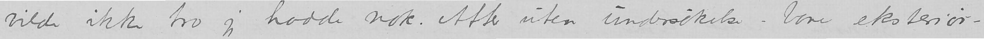vilde ikke tro jeg hadde nok. Atter uten undersøkelse – bare eksteriør-
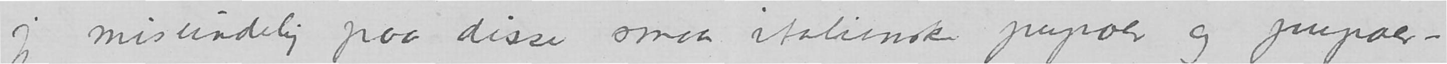jeg misundelig paa disse smaa italienske pupoer og pupaer –
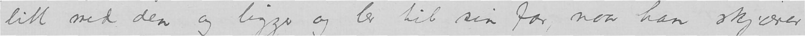litt med den og ligger og ler til sin far, naar han skjærer
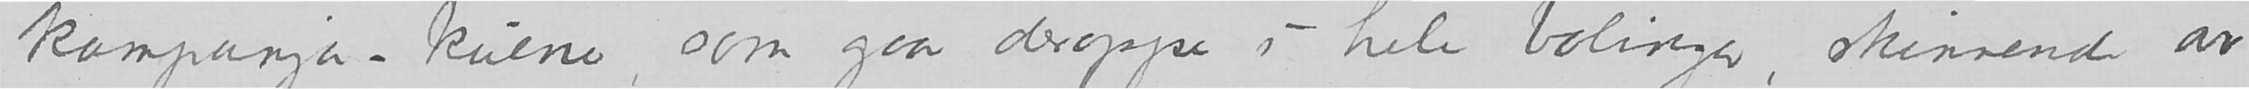kampanja-kuene, som gaar deroppe i hele bølinger, skinnende av
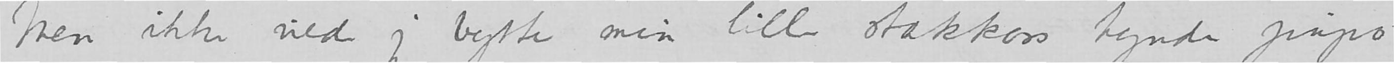Men ikke vilde jeg bytte min lille stakkars tynde pupo –
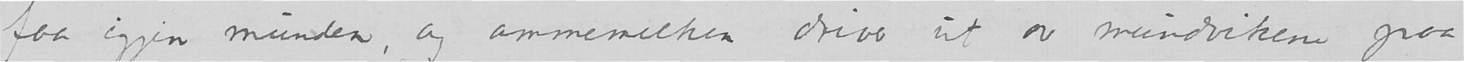faa igjen munden, og ammemelken driver ut av mundviken paa
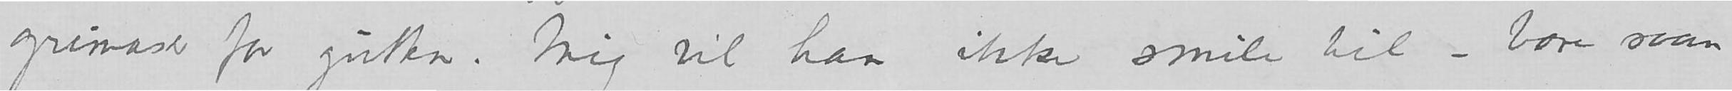grimaser for gutten. Mig vil han ikke smile til – bare saan
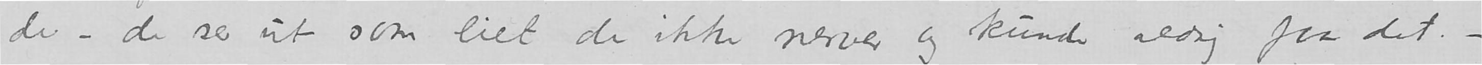de – de ser ut som eiet de ikke nerver og kunde aldrig faa det. –
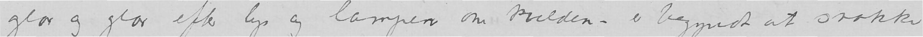glor og glor efter lys og lampen om kvelden – er begyndt at snakke
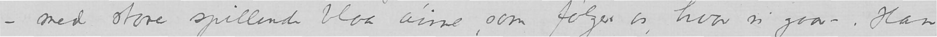med store spillende blaa øine, som følger os, hvor vi gaar -. Han
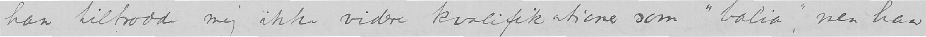han tiltrodde mig ikke videre kvalifikationer som «balia», men han
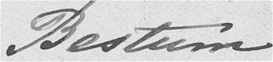Bestum
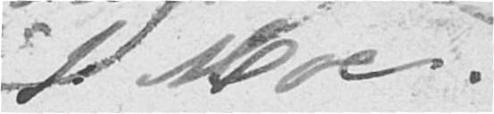f Moe.
